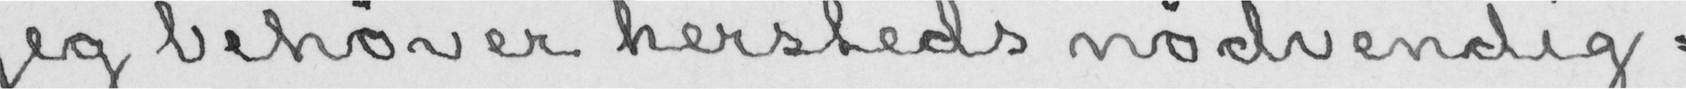Jeg behøver hersteds nødvendig-
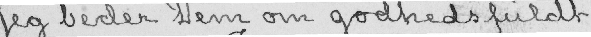Jeg beder Dem om godhedsfuldt
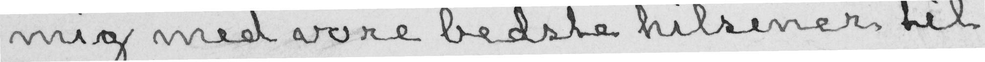mig med vore bedste hilsener til
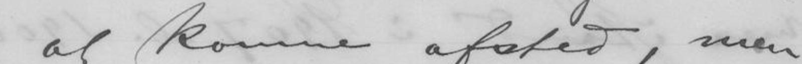at komme afsted, men
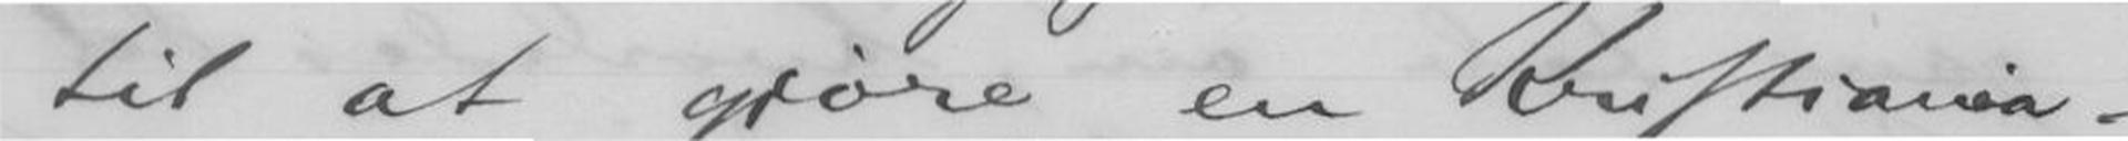til at gjøre en Kristiania-
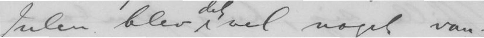Julen blev det vel noget van-
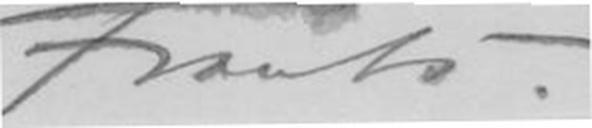Frants.
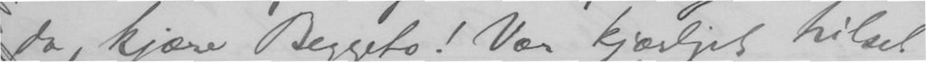da, kjære Beggeto! Vær kjærligt hilset
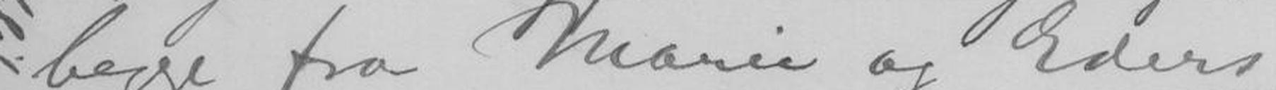begge fra Marie og Eders
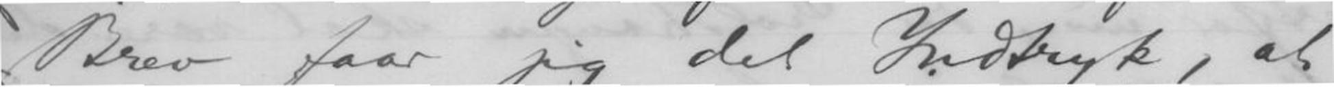Brev faar jeg det Indtryk, at
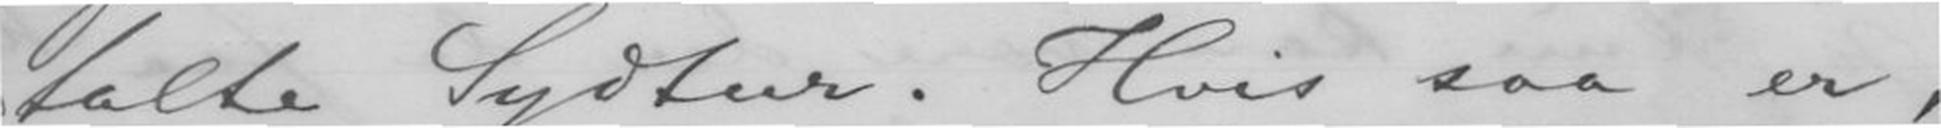talte Sydtur. Hvis saa er,
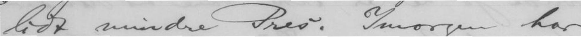lidt mindre Pres. Imorgen har
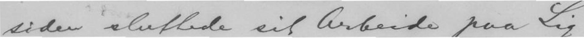siden sluttede sit Arbeide paa Lig-
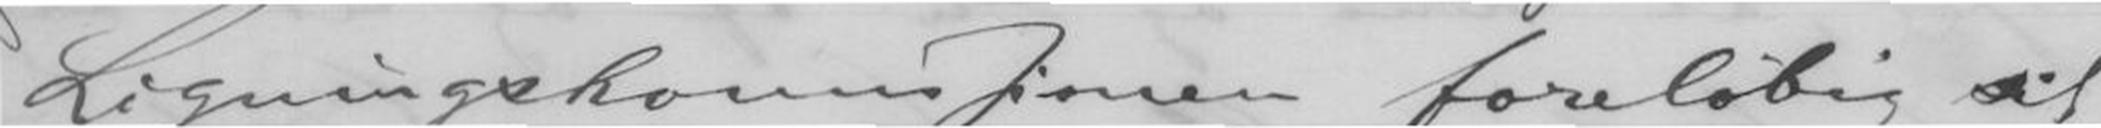Ligningskomissionen foreløbig sit
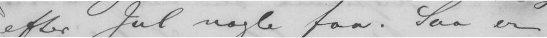efter Jul nogle faa. Saa er
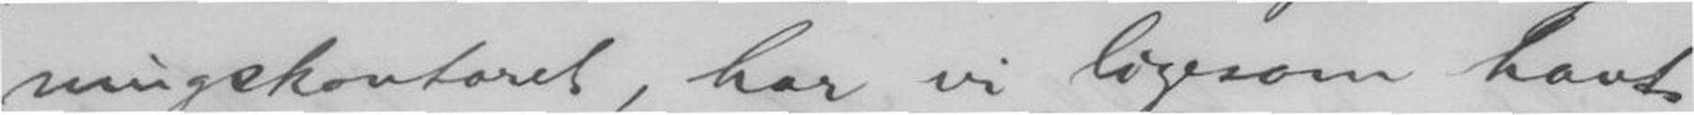ningskontoret, har vi ligesom havt
